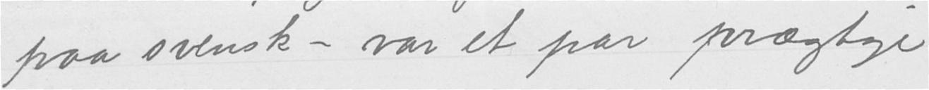paa svensk - var et par prægtige
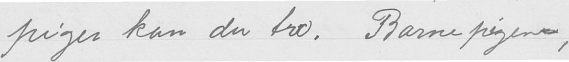piger kan du tro. Barnepigen,
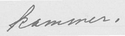kammer.
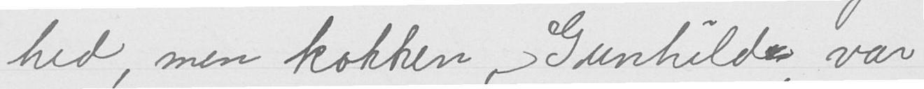hed, men kokken, Gunhild, var
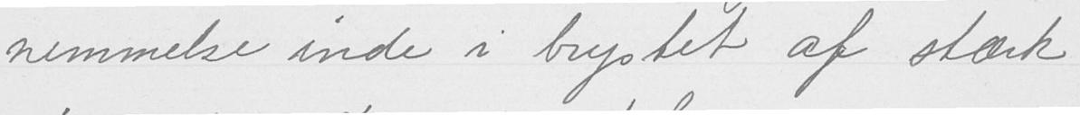nemmelse inde i brystet af stærk
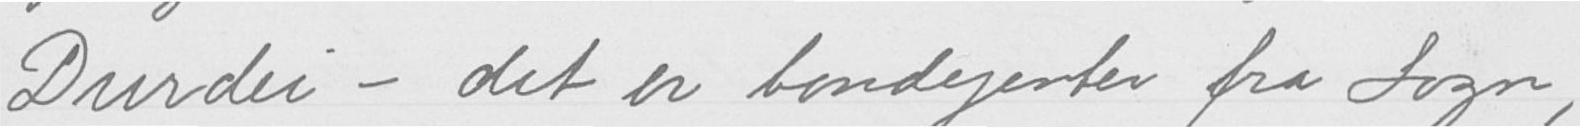Durdei - det er bondejenter fra Sogn,
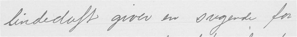lindeduft giver en sugende for-
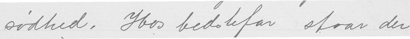sødhed. Hos bedstefar staar der
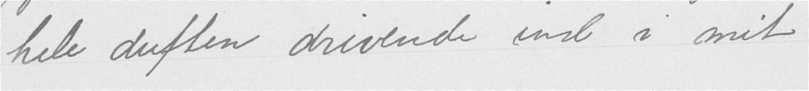hele duften drivende ind i mit
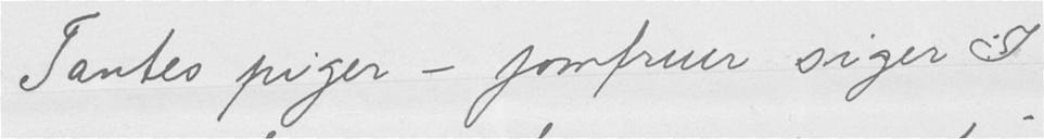Tantes piger - jomfruer siger I
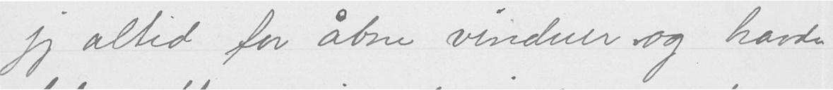jeg altid for aabne vinduer og havde
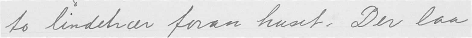to lindetrær foran huset. Der laa
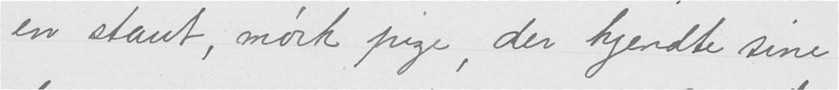en staut, mørk pige, der kjendte sine
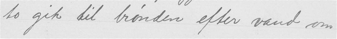to gik til brønden efter vand om
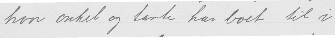hvor onkel og tante har boet til i-
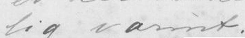lig varmt.-
